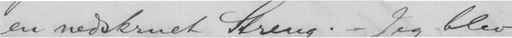en nedskrevet Streng. - Jeg blev
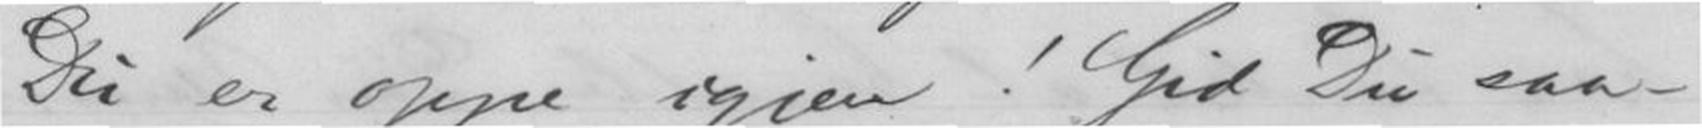Du er oppe igjen! Gid Du saa-
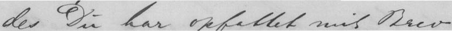des Du har opfattet mit Brev.
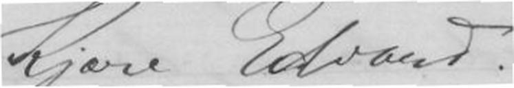Kjære Edvard!
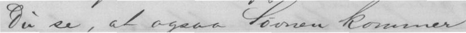skal Du se, at ogsaa Søvnen kommer
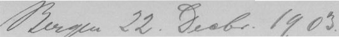Bergen 22. Decbr. 1903.
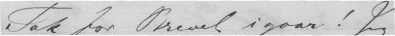Tak for Brevet igaar! Jeg
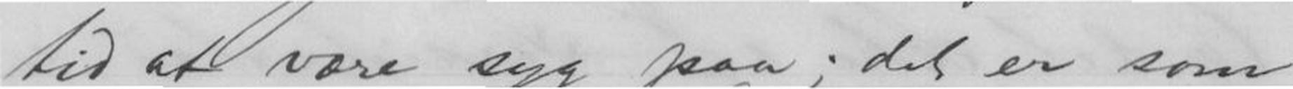tid at være syg paa; det er som
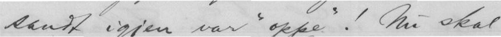sandt igjen var "oppe"! Nu
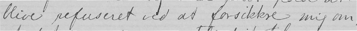blive refuseret ved at forsikkre mig om,
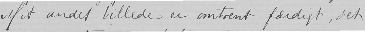Mit andet billede er omtrent færdigt, det
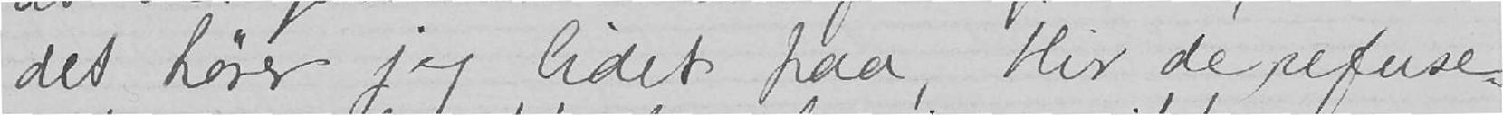det hører jeg lidet paa, blir de refuse-
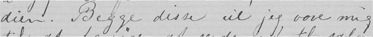dien. Begge disse vil jeg vove mig
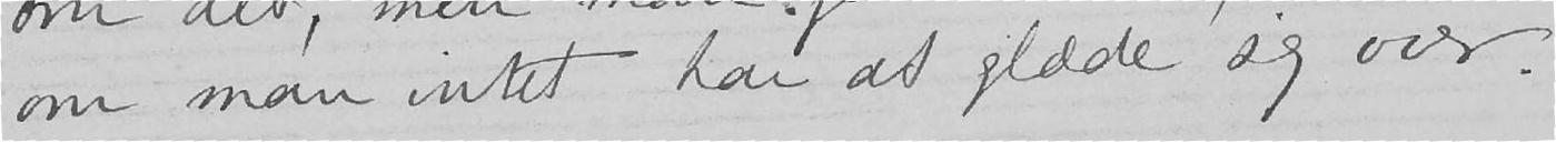om man intet har at glæde sig over.
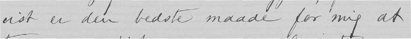vist er den bedste maade for mig at
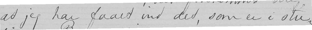at jeg har faaet ind det, som er i stu-
